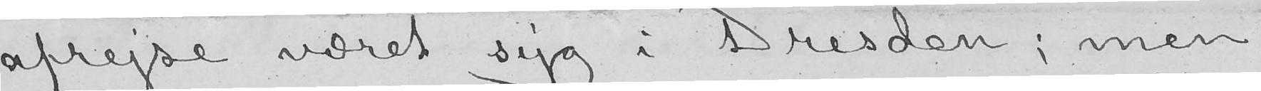afrejse været syg i Dresden; men
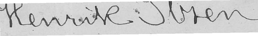Henrik Ibsen.
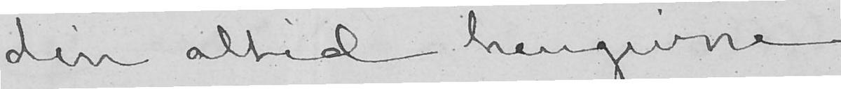din altid hengivne
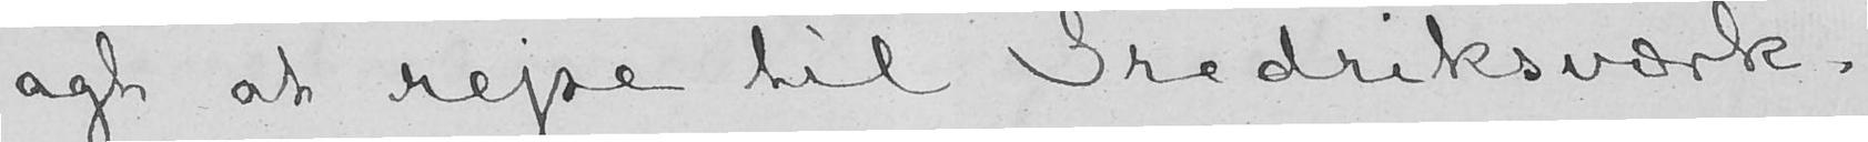agt at rejse til Fredriksværk.
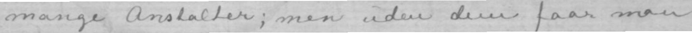mange Anstalter; men uden dem faar man
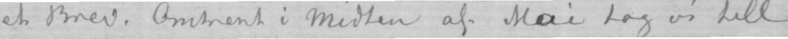et Brev. Omtrent i Midten af Mai tog vi till
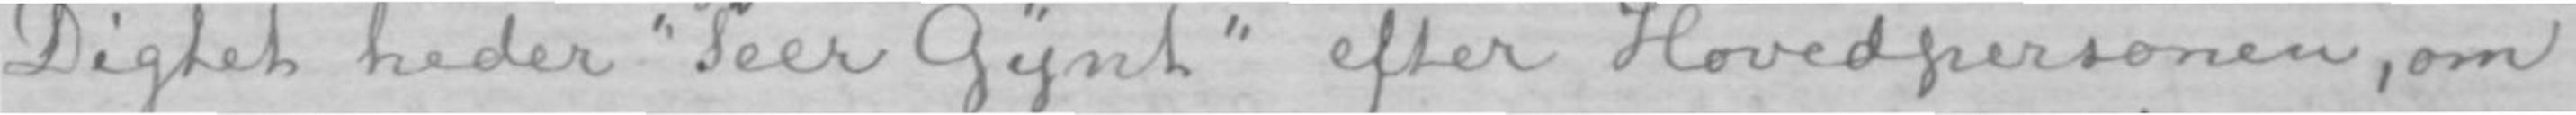Digtet heder «Peer Gynt» efter Hovedpersonen, om
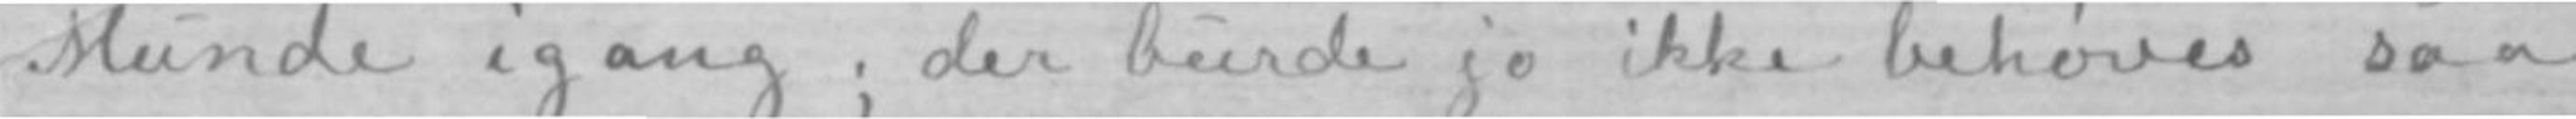Munde igang; der burde jo ikke behøves saa
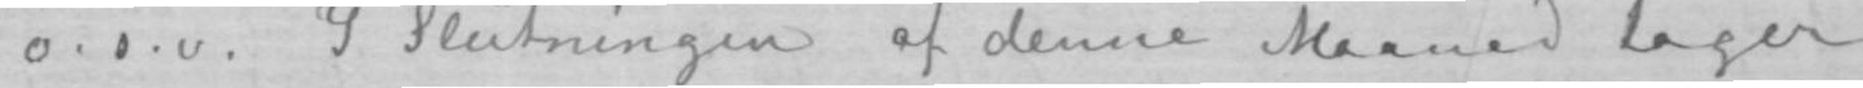o. s. v. I Slutningen af denne Maaned tager
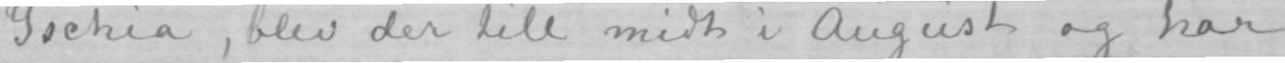Ischia, blev der till midt i August og har
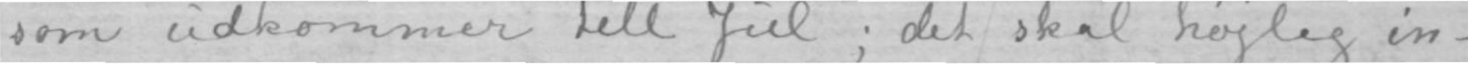som udkommer till Jul; det skal højlig in-
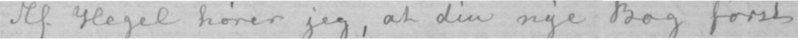Af Hegel hører jeg, at din nye Bog først
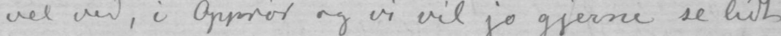vel ved, i Opprør og vi vil jo gjerne se lidt
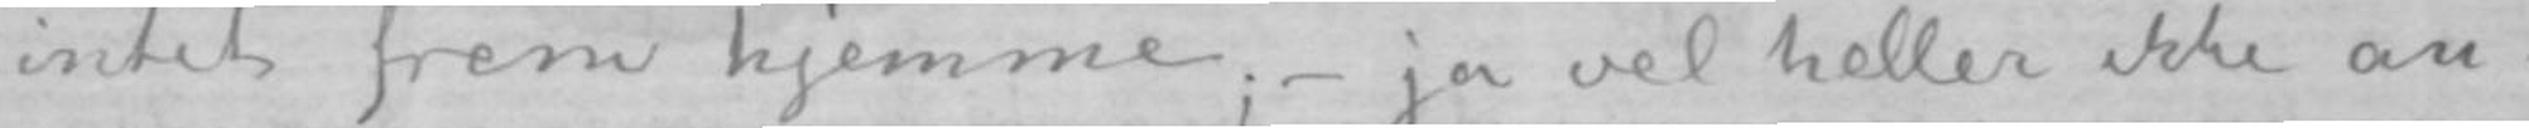intet frem hjemme;- ja vel heller ikke an-
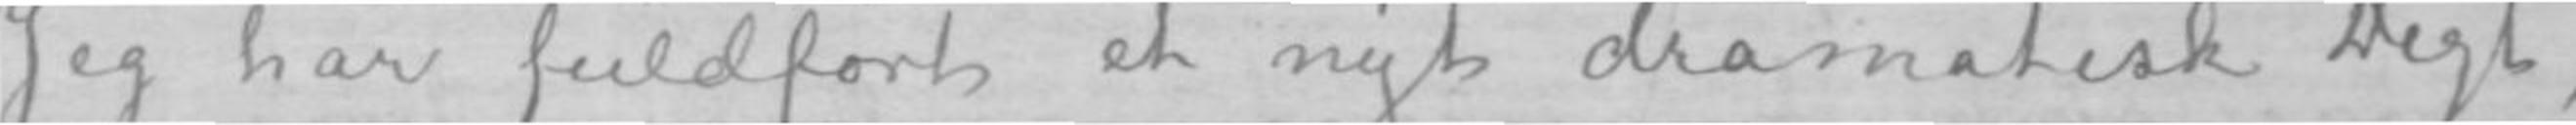Jeg har fuldført et nyt dramatisk Digt,
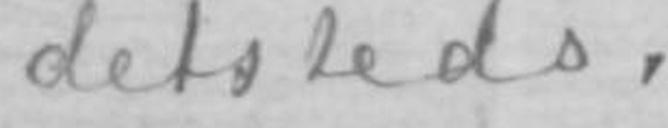detsteds.
